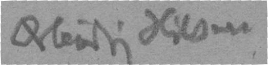Ærbødig Hilsen
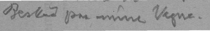Besked paa mine Vegne.
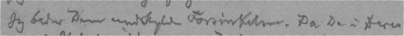Jeg beder Dem undskylde Forsinkelsen. Da De i Deres
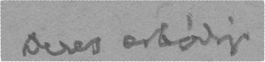Deres ærbødige
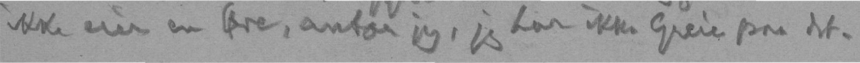ikke eier en Øre, antar jeg, jeg har ikke Greie paa det.
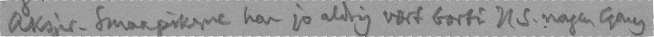Aksjer. Smaapikerne har jo aldrig vært borti NS. nogen Gang
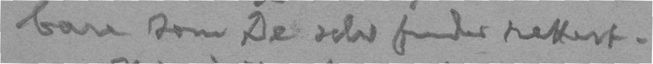bare som De selv finder rettest.
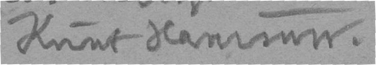Knut Hamsun.
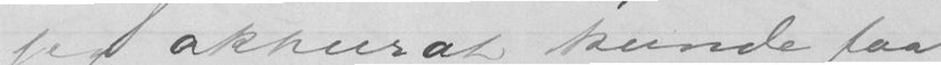jeg akkurat kunde faa
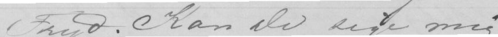Fryd. Kan De sige mig
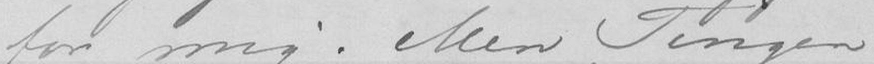for mig. Men Tingen
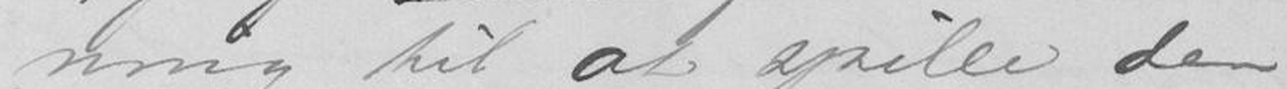ning til at spille den
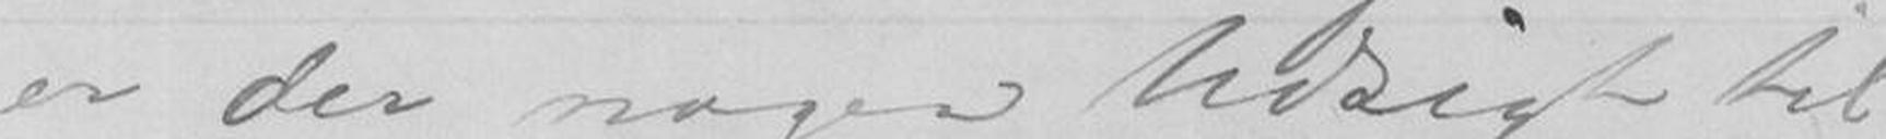er der nogen Udsigt til
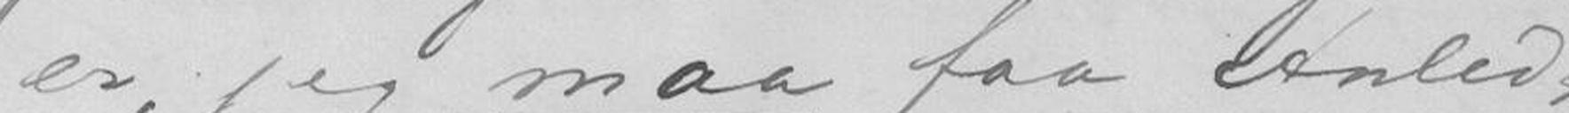er, jeg maa faa Anled-
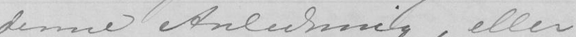denne Anledning, eller
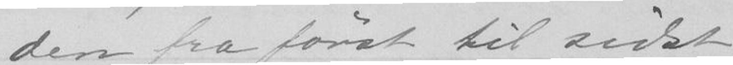den fra først til sidst
